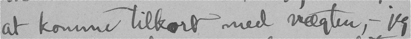at komme tilkort med vægten, - jeg
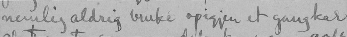nemlig aldrig brerke opigjen et gangkar
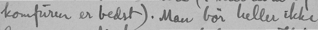komfuren er bedst). Man bør heller ikke
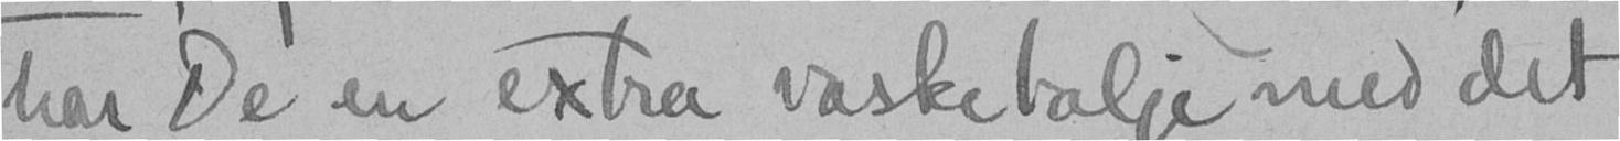har De en extra vaskebalje med det
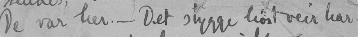De var her. - Det stygge høst veir har
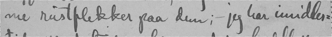me rustflekker paa dem; - jeg har imidler-
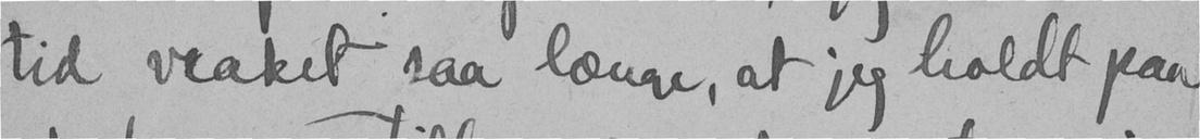tid vraket saa længe, at jeg holdt paa
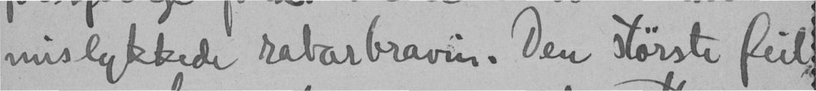mislykkede rabarbravin. Den største feil
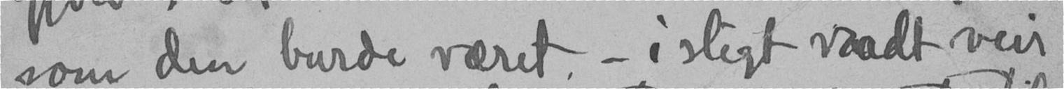som den burde været, - i sligt vaadt veir
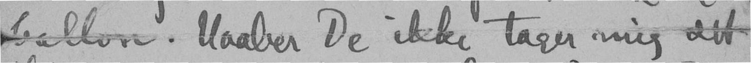ballon. Haaber De ikke tager mig det
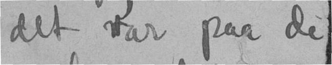det var paa di
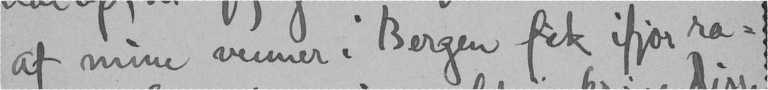af mine venner i Bergen fik ifjor ra-
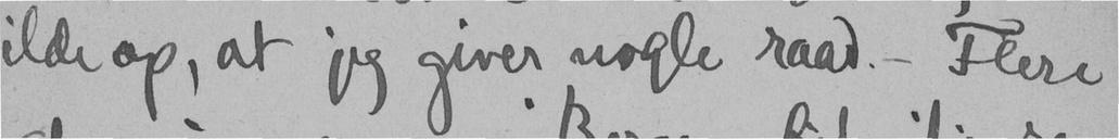ilde op, at jeg giver nogle raad.- Flere
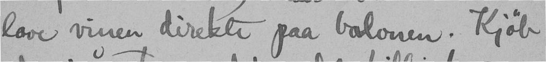lave vinen direkte paa balonen. Kjøb
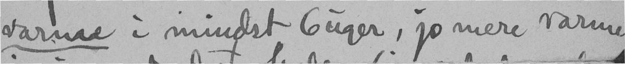varme i mindst 6 uger, jo mere varme
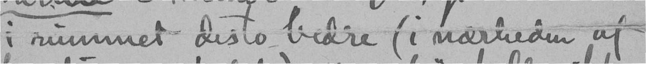i rummet desto bedre (i nærheden af
